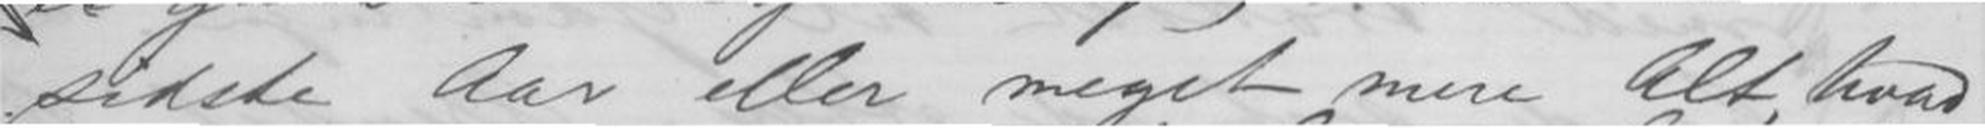sidste Aar eller meget mere Alt, hvad
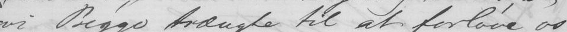vi Begge trængte til at forlove os
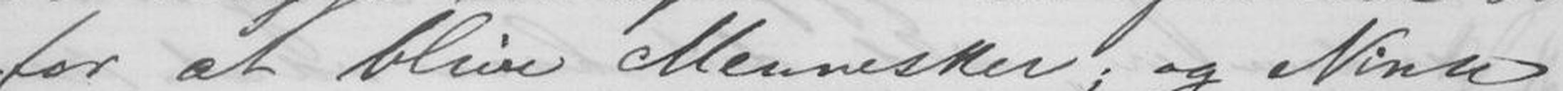for at blive Mennesker; og Nina
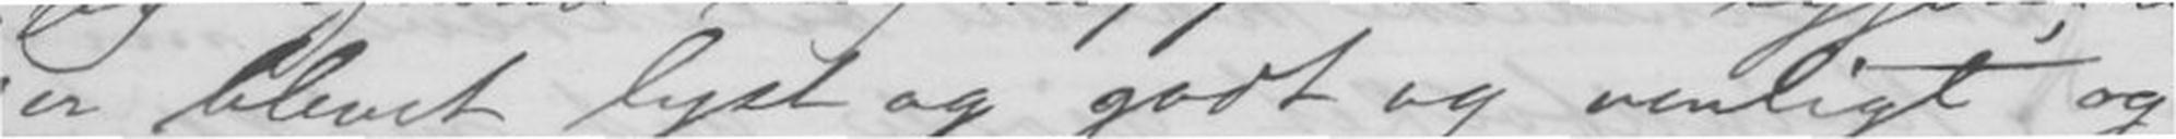er blevet lyst og godt og venligt og
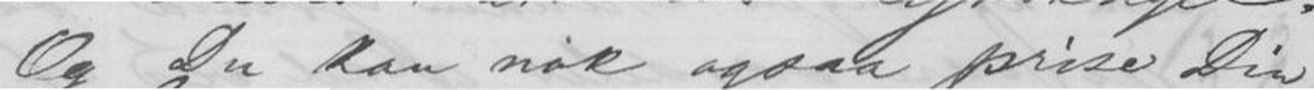Og Du kan nok ogsaa prise Din
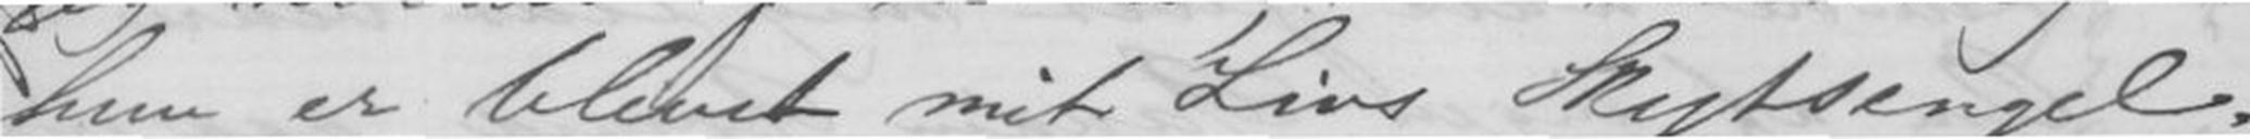hun er blevet mit Livs Skytsengel.
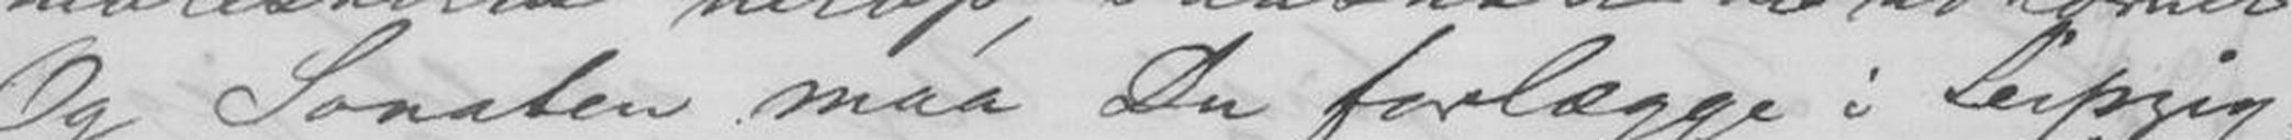Og Sonaten maa Du forlægge i Leipzig,
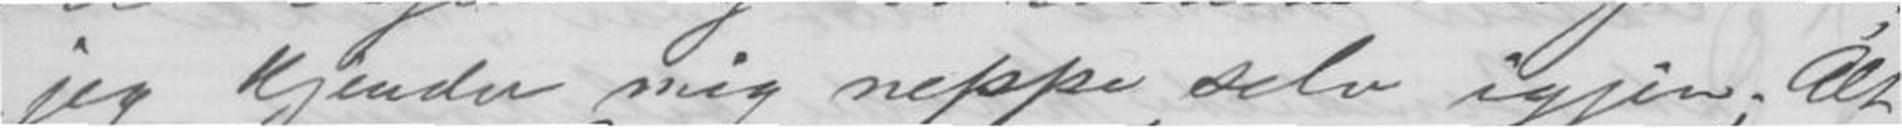jeg kjender mig neppe selv igjen; Alt
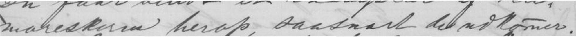moreskerne herop, saasnart de udkommer.
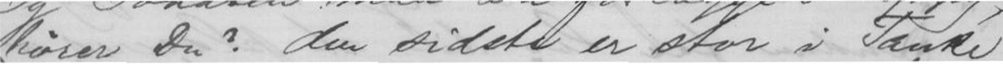hører Du? Den sidste er stor i Tanke
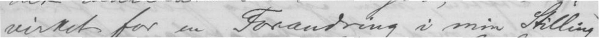virket for en Forandring i min Stilling
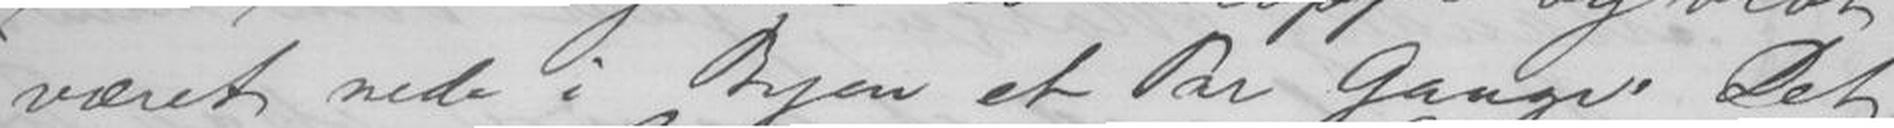været nede i Byen et Par Gange. Det
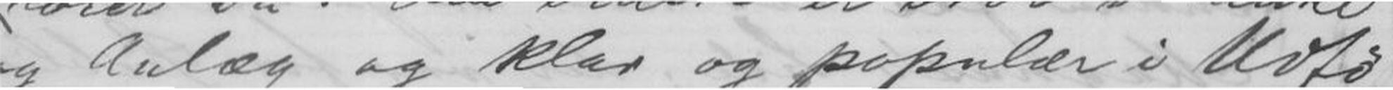og Anlæg og klar og populær i Udfø-
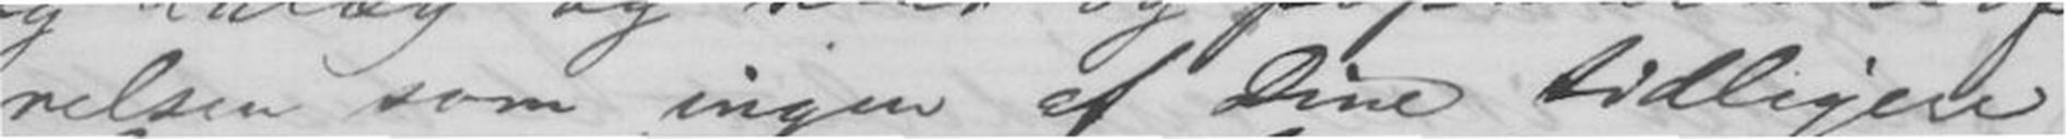relsen som ingen af Dine tidligere
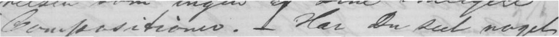Compositioner. - Har Du seet noget
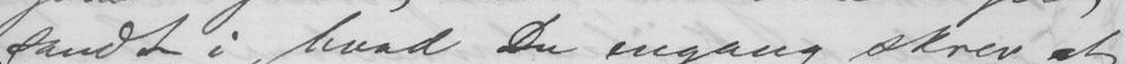sandt i, hvad Du engang skrev, at
